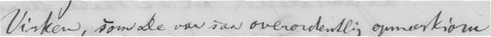Virken, som De var saa overordentlig opmærksom
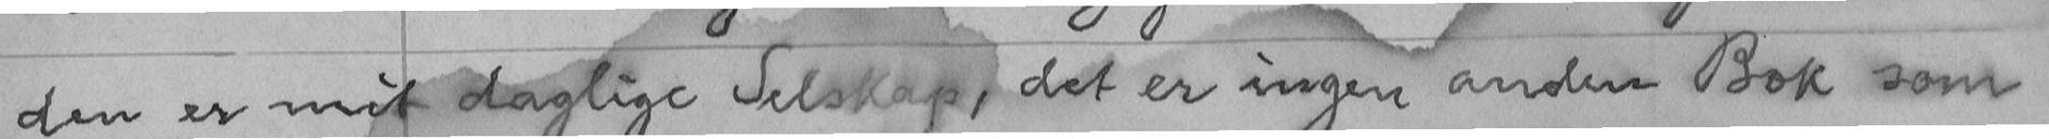den er mit daglige Selskap, det er ingen anden Bok som
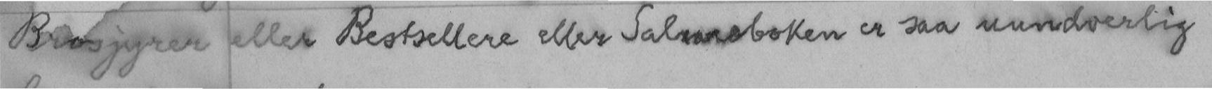Brosjyrer eller Bestsellere eller Salmeboken er saa uundverlig
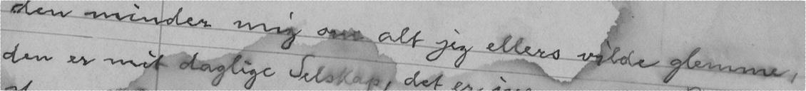den minder mig om alt jeg ellers vilde glemme,
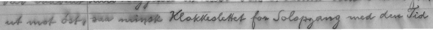ut mot øst, saa minsk Klokkeslettet for Solopgang med den Tid
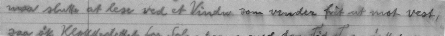maa slutte at lese ved et Vindu som vender frit ut mot vest,
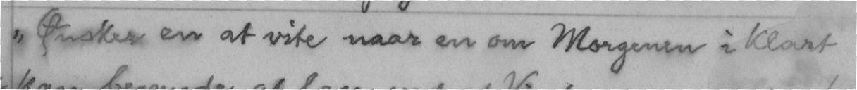"Ønsker en at vite naar en om Morgenen i klart
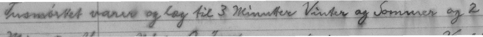Tusmørket varer og læg til 3 Minutter Vinter og Sommer og 2
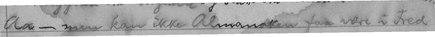Aa - men kan ikke Almanaken faa være i Fred
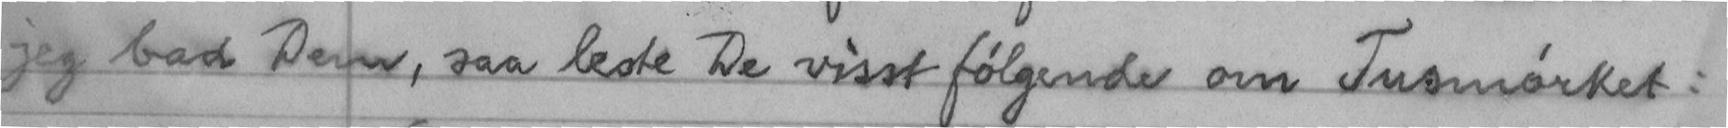jeg bad Dem, saa leste De visst følgende om Tusmørket:
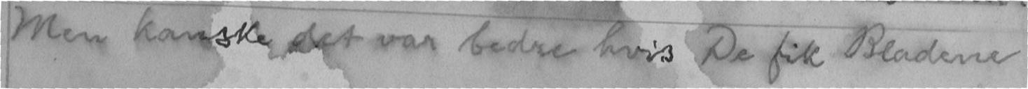Men kanske det var bedre hvis De fik Bladene
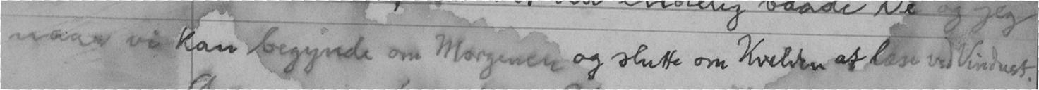naar vi kan begynde om Morgenen og slutte om Kvelden at læse ved Vinduet.
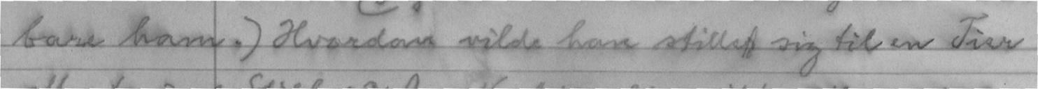bare ham.) Hvordan vilde han stillet sig til en Tier
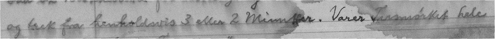og trek fra henholdsvis 3 eller 2 Minutter. Varer Tusmørket hele
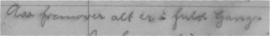Aar fremover alt er i fuld Gang.
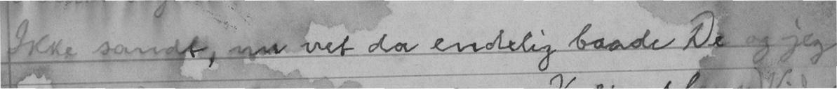Ikke sandt, nu vet da endelig baade De og jeg
